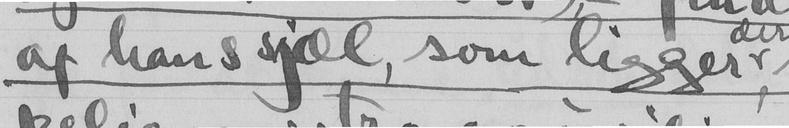af han sjæl, som ligger
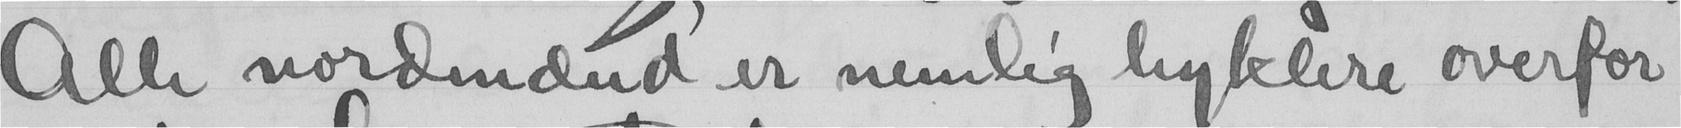Alle nordmænd er nemlig hyklere overfor
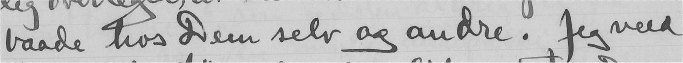baade hos Dem selv og andre. Jeg veed
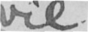vil
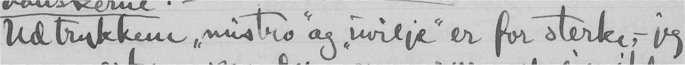tidtrukkene "mistro" og "uvilje" er for sterke, - jeg
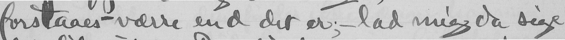forstaaes - værre end det er - lad mig da sige
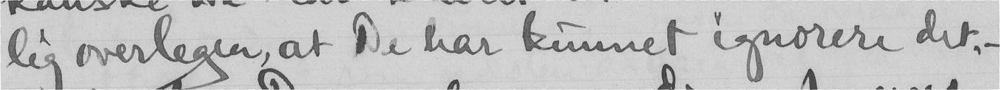lig overlegen, at De har kunnet ignorere det, -
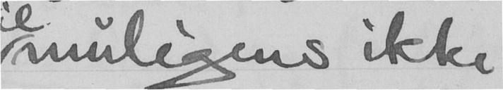muligens ikke
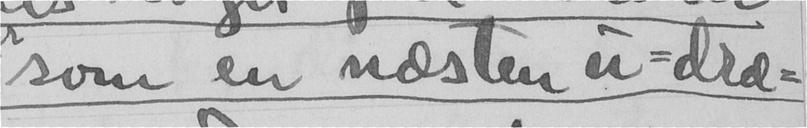som en næsten u-dræ-
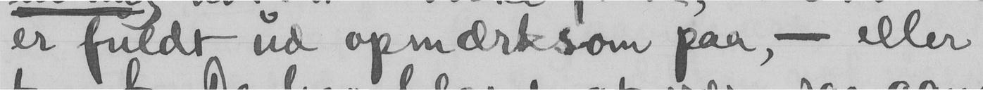er fuldt ud opmærksom paa, - eller
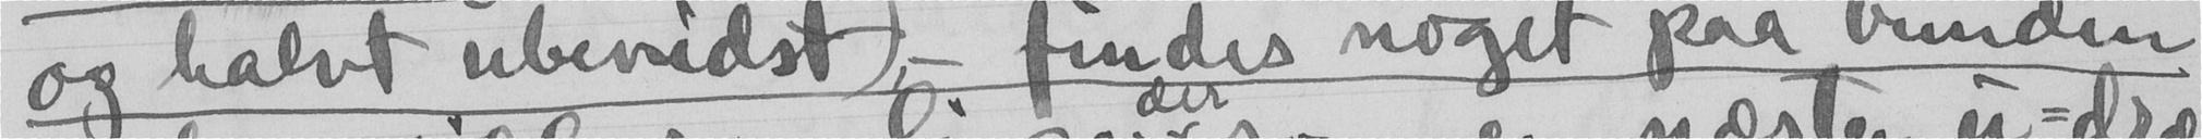og halvt ubevidst), - findes noget paa bunden
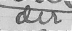der
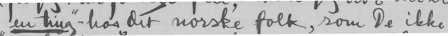"en ting" - hos det norske folk, som De ikke
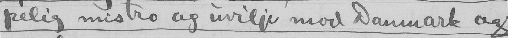pelig mistro og uvilje mod Danmark og
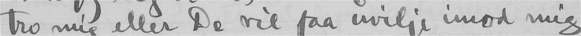tro mig eller De vil faa uvilje imod mig
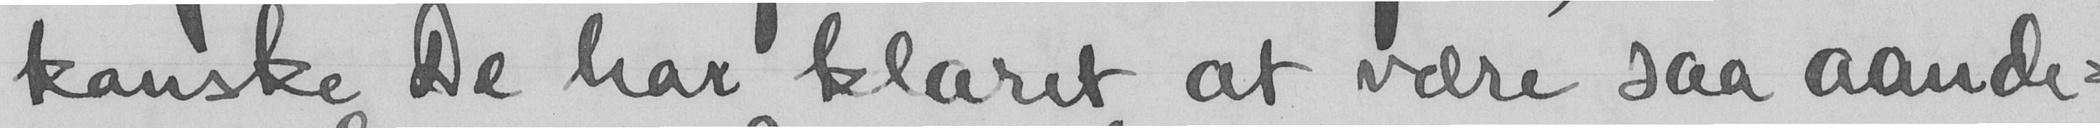kanske De har klaret at være saa aande-
